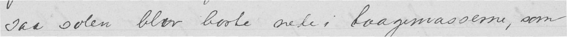saa solen blev borte nede i taagemasserne, som
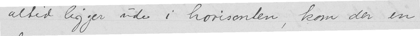altid ligger ude i horisonten, kom der en
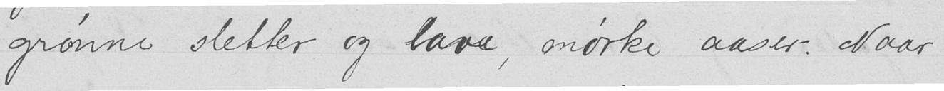grønne sletter og lave, mørke aaser. Naar
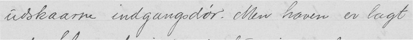udskaarne indgangsdør. Men haven er lagt
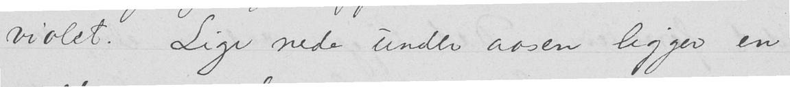violet. Lige nede under aasen ligger en
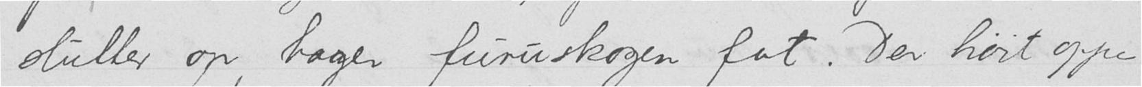slutter op, tager furuskogen fat. Der høit oppe
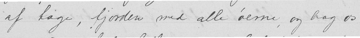af tage, fjorden med alle øerne, og bag os
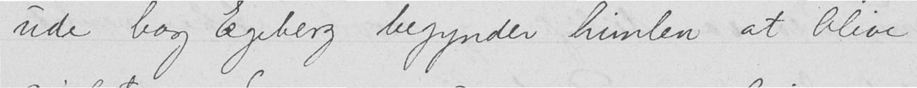ude bag Egeberg begynder himlen at blive
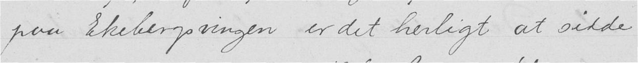paa Ekebergsvingen er det herligt at sidde
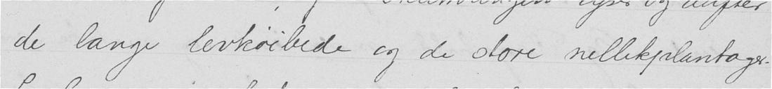de lange levkøibede og de store nellikplantager.
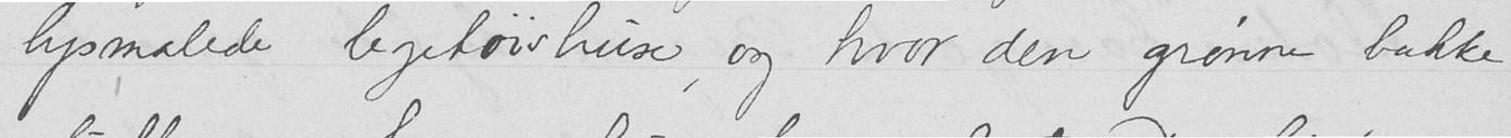lysmalede legetøishuse, og hvor den grønne bakke
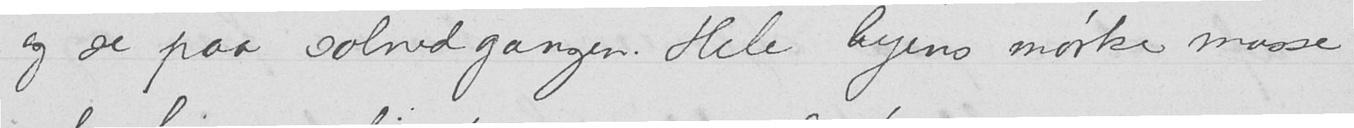og se paa solnedgangen. Hele byens mørke masse
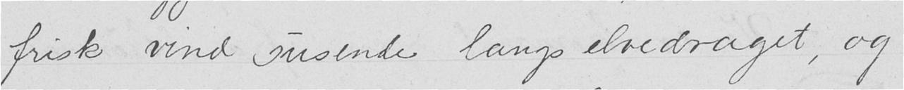frisk vind susende langs elvedraget, og
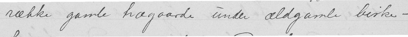række gamle trægaarde under ældgamle birke-
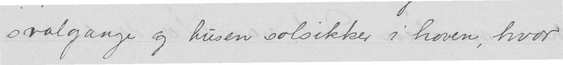svalgange og tusen solsikker i haven, hvor
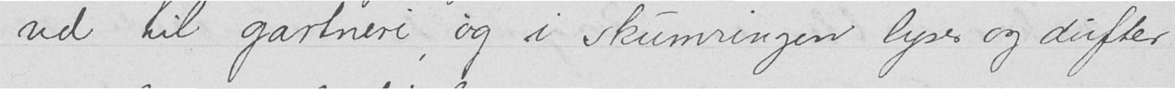ud til gartneri, og i skumringen lyser og dufter
